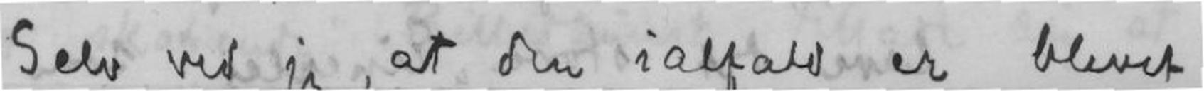Selv ved jeg, at den ialfald er blevet
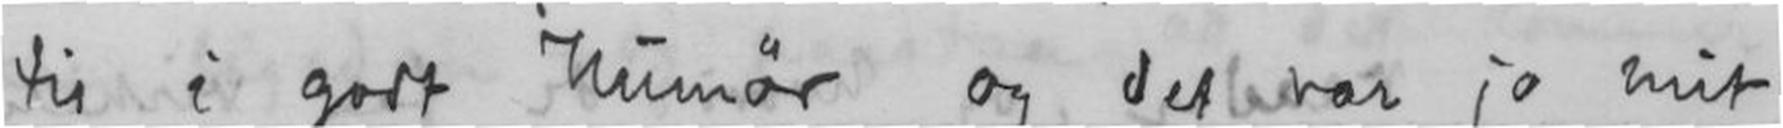til i godt Humør og det var jo mit
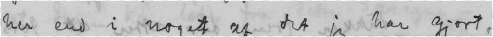her end i noget af det jeg har gjort,
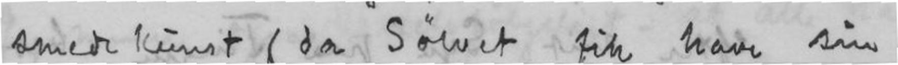smedkunst (da Sølvet fik have sin
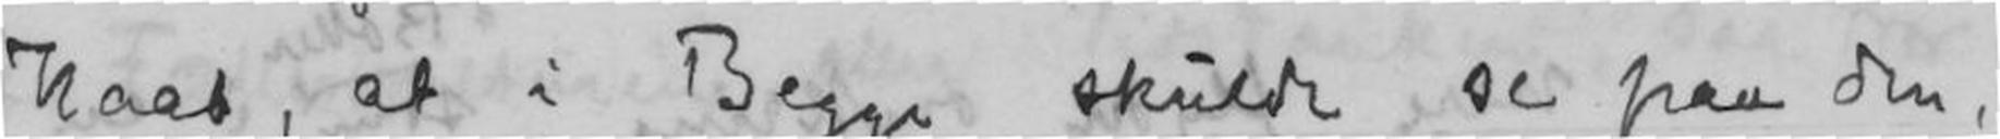Haab, at i Begge skulde se paa den,
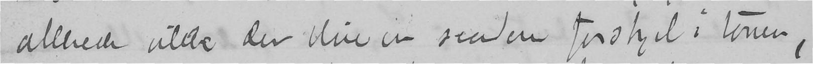allerede vilde der blive en saaden forskjel i tonen,
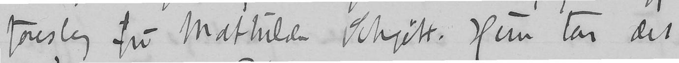foreslog fru Mathilde Schjøtt. Hun tar det
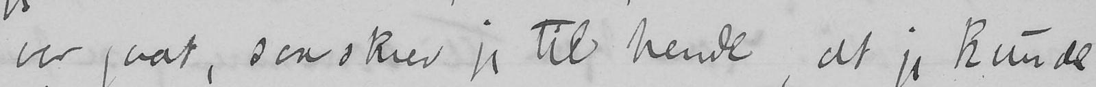var gaat, saa skrev jeg til hende, at jeg kunde
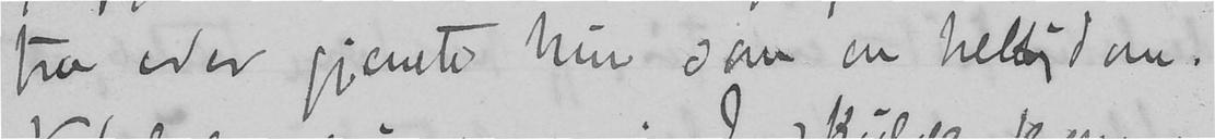fra eder gjemte hun som en helligdom.
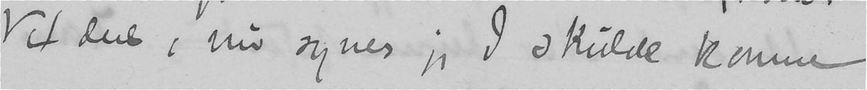Vet dere, nu synes jeg I skulde komme
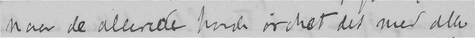naar de allerede havde ordnet det med alle
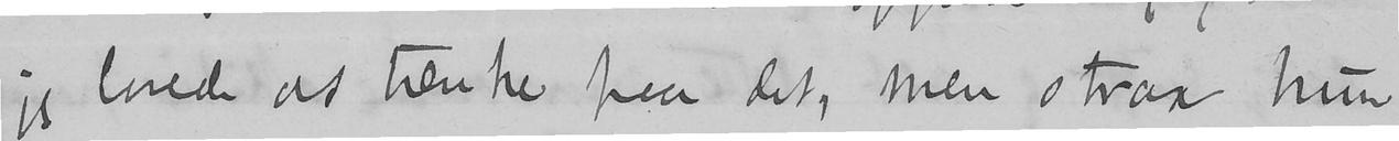jeg lovede at tænke paa det, men strax hun
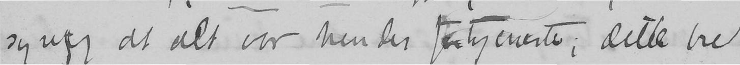synlig at alt var hendes fortjeneste; dette brev
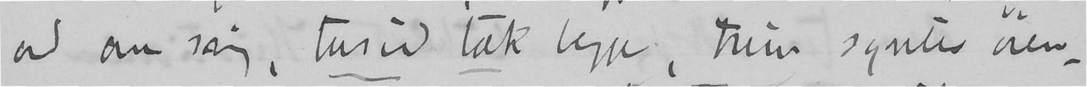ord om mig, tusind tak begge, hun syntes øien-
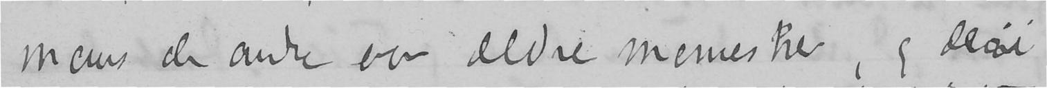mens de andre var ældre mennesker, og deri
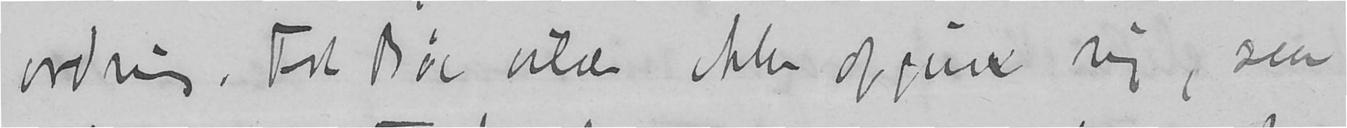ordning. Frk Bøe vilde ikke opgive sig, saa
